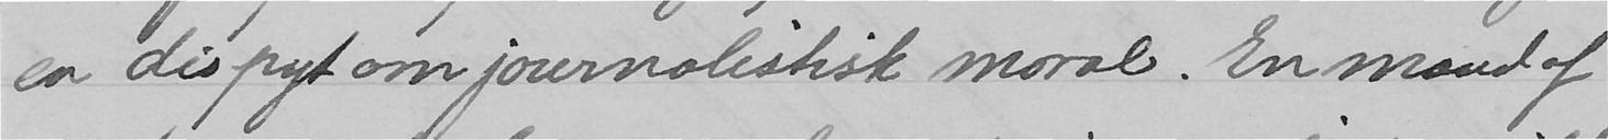en dispyt om journalistisk moral. En mand af
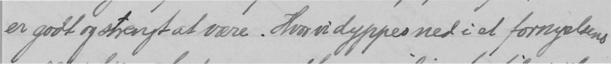er godt og strengt at være. Hvor vi dyppes ned i et fornyelsens
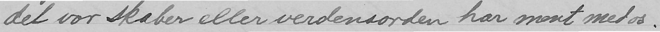det vor skaber eller verdensorden har ment med os.
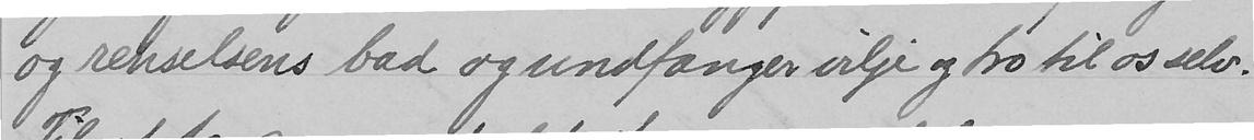og renselsens bad og undfanger vilje og tro til os selv.
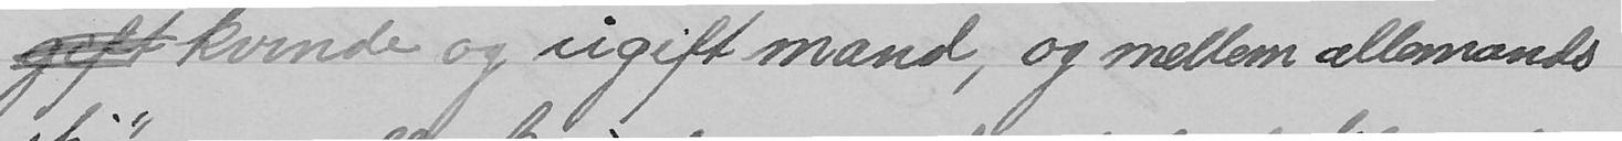gift kvinde og ugift mand, og mellem allemands
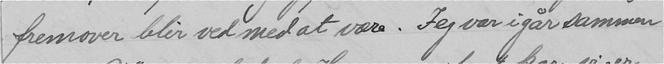fremover blir ved med at være. Jeg var igår sammen
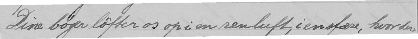Dine bøger løfter os op i en ren luft, i en sfære, hvor der
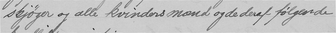skjøger og alle kvinders mænd og de deraf følgende
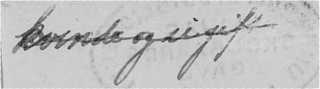kvinde og ugift -
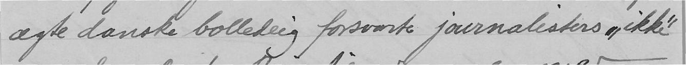ægte danske bolledeig forsvarte journalisters "ikke"
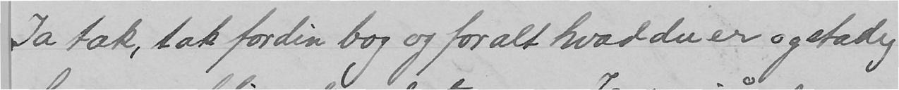Ja tak, tak for din bog og for alt hvad du er og stadig
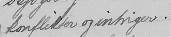konflikter og intriger.
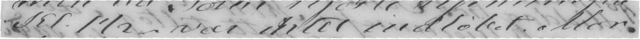Kl 1½ var Intet indløbet. Mor
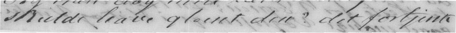skulde have glemt den? det fortjente
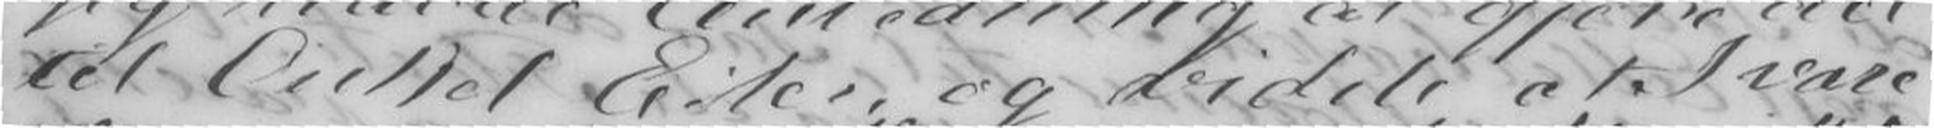til Onkel Eiler, og vidste at I vare
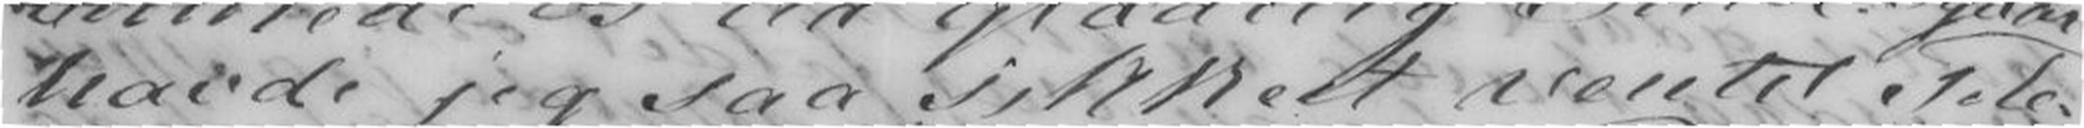havde jeg saa sikkert ventet Tele-
